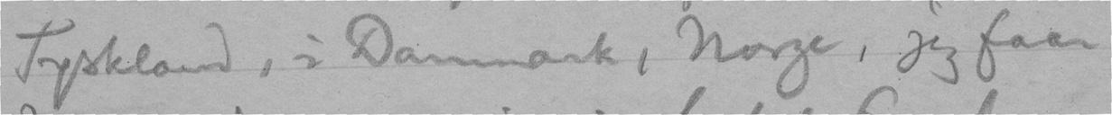Tyskland, i Danmark, Norge, jeg faar
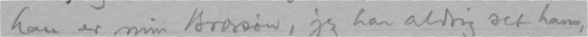han er min Brorsøn, jeg har aldrig set ham,
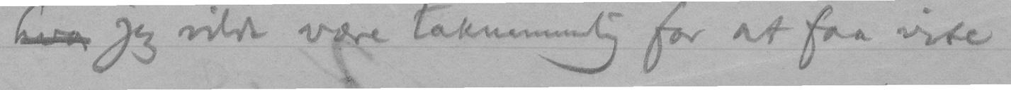hva jeg vilde være taknemmelig for at faa vite
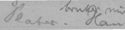Plater. Han
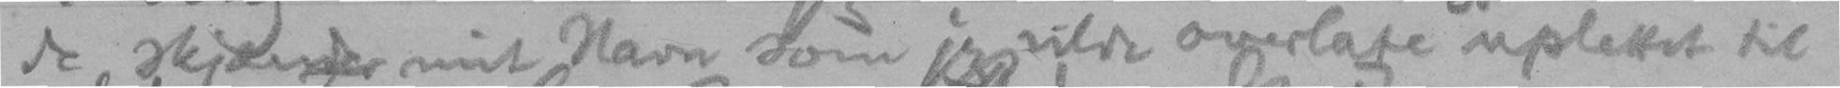de skjænder mit Navn som jeg vilde overlate uplettet til
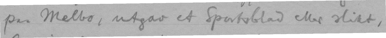paa Melbo, utgav et Sportsblad eller slikt,
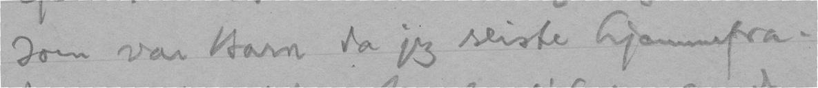som var Barn da jeg reiste hjemmefra.
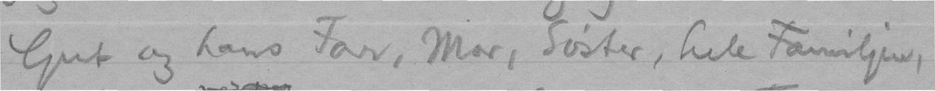Gut og hans Far, Mor, Søster, hele Familjen,
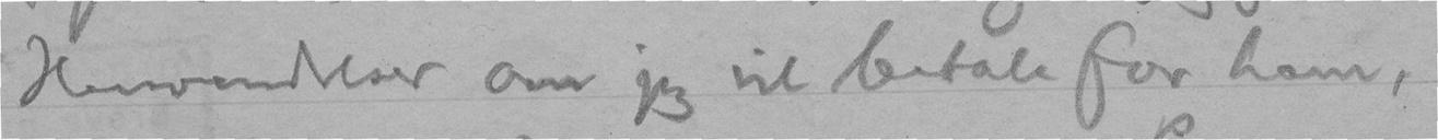Henvendelser om jeg vil betale for ham,
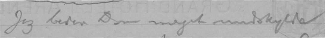Jeg beder Dem meget undskylde
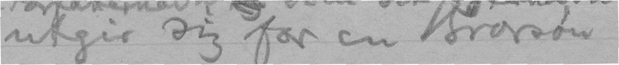utgir sig for en Brorsøn
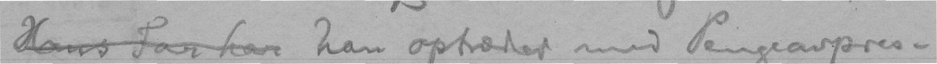Hans Far har han optræder med Pengeavpres-
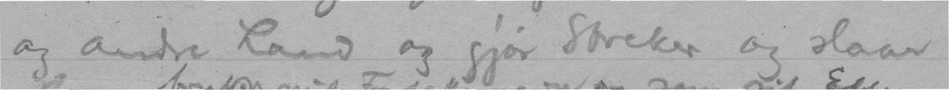og andre Land og gjør Streker og slaar
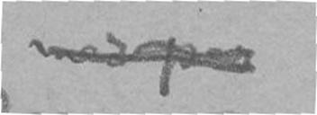modpaa
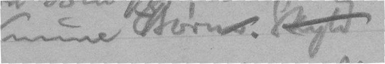mine Børns. Skyld
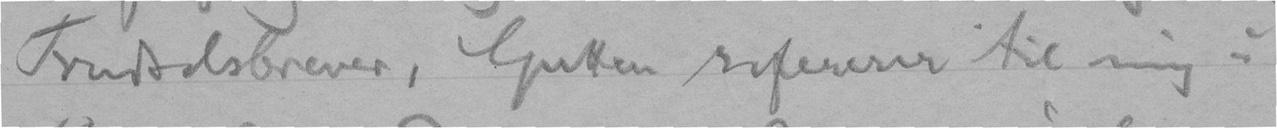Trudselsbrever, Gutten refererer til mig i
